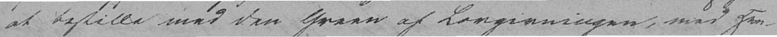at bestille med den Green af Lovgivningen, med Hen-
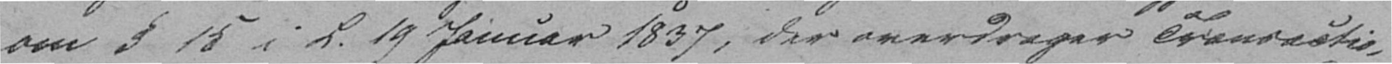om § 15 i L. 19 Januar 1837, der overdrager Transactio-
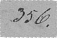356.
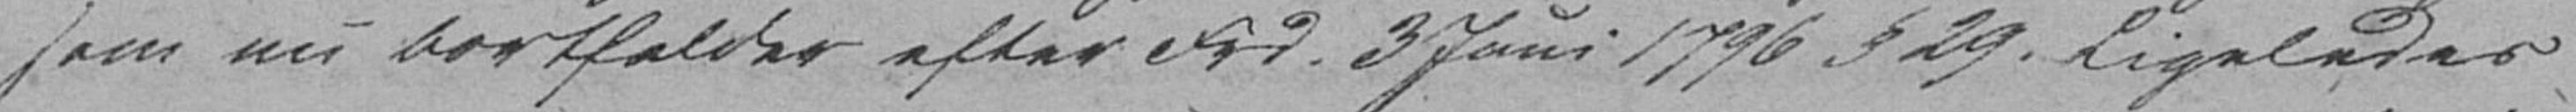som nu bortfalder efter Frd. 3 Juni 1796 § 29. Ligeledes
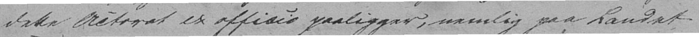dette Actorat ex officio paaligger, nemlig paa Landet
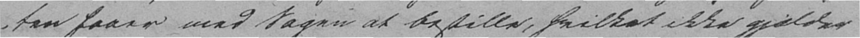ten faaer med Sagen at bestille, hvilket ikke gjælder
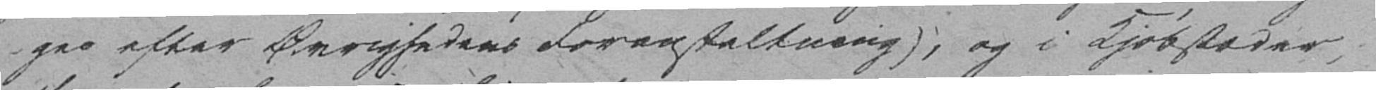ges efter Øvrighedens Foranstaltning), og i Kjøbstæder,
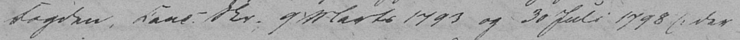Fogden, Canc. Skr. 9 Marts 1793 og 30 Juli 1798 (: der
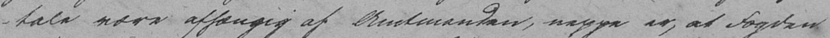tale være afhængig af Amtmanden, neppe er, at Fogden
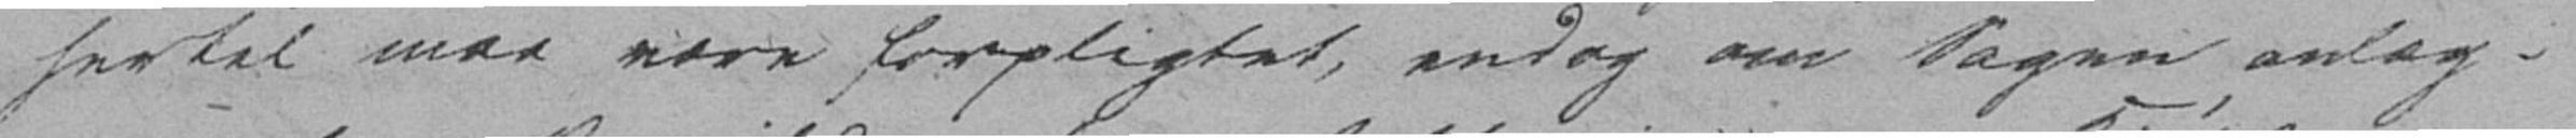hertil maa være forpligtet, endog om Sagen anlæg-
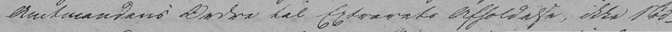Amtmandens Ordre til Extrarets Afholdelse, ikke Nød-
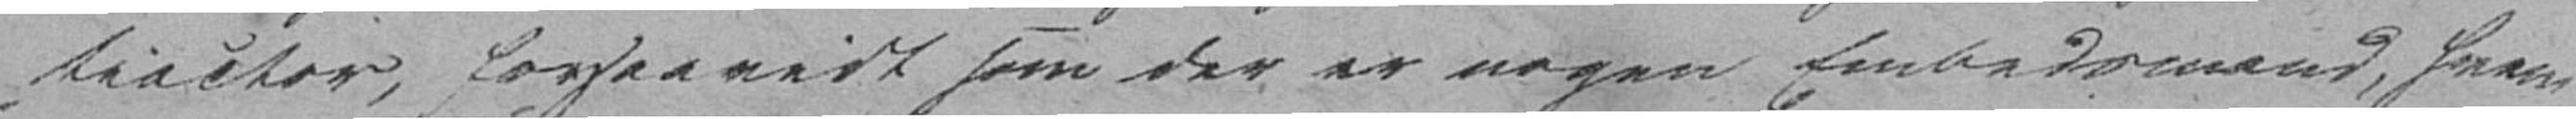tiactor, forsaavidt som der er nogen Embedsmand, hvem
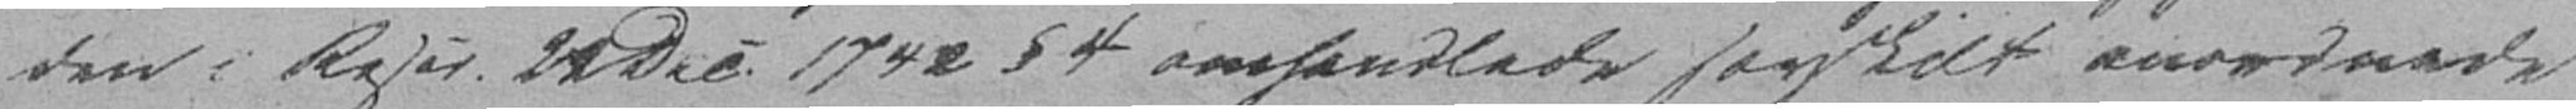den i Rescr. 22 Dec. 1742 § 4 omhandlede særskilt anordnede
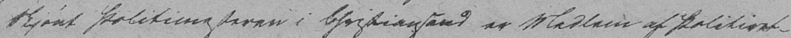Skjønt Politimesteren i Christiansand er Medlem af Politiret-
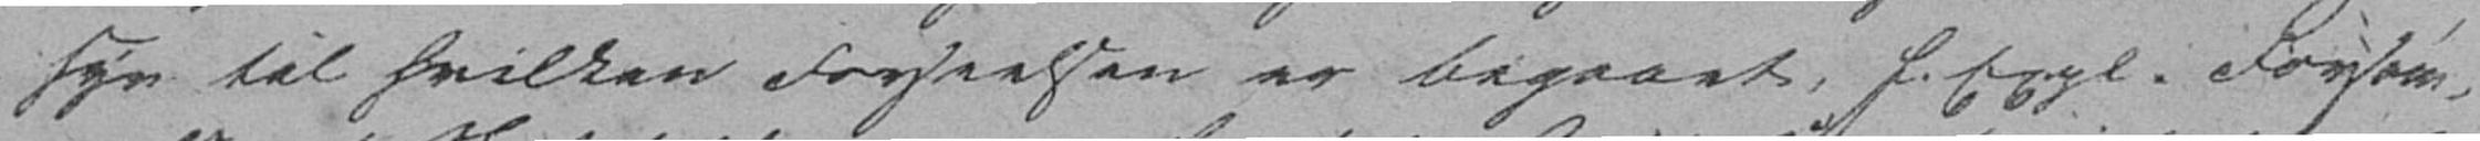syn til hvilken Forseelsen er begaaet, f. Expl. Forsøm-
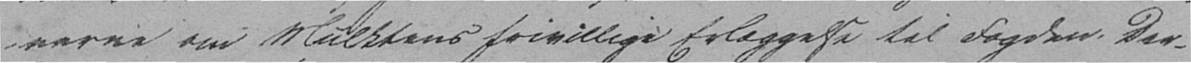nerne om Mulktens frivillige Erlæggelse til Fogden. Der-
